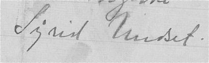Sigrid Undset.
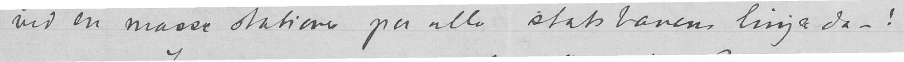ved en masse stationer paa alle statsbanens linjer da – !
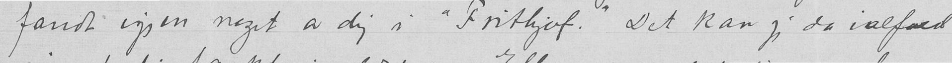fandt igjen noget av dig i "Frithjof." Det kan jeg da ialfald
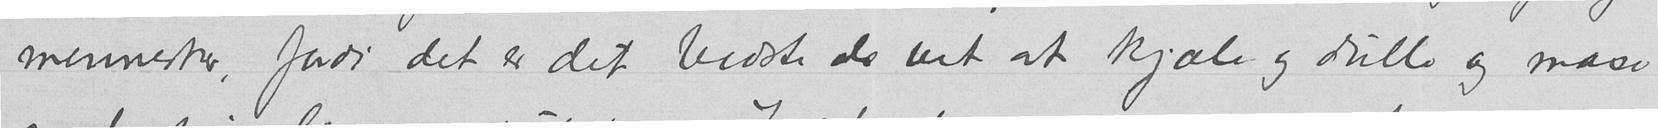mennesker, fordi det er det bedste de vet at kjæle og dulle og mase
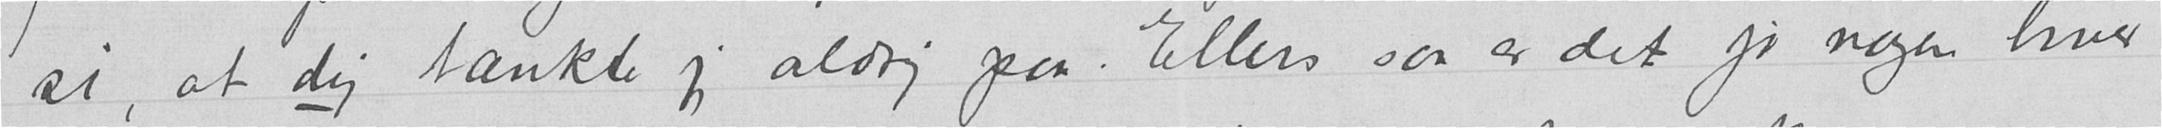si, at dig tænkte jeg aldrig paa. Ellers saa er det jo nogen hver
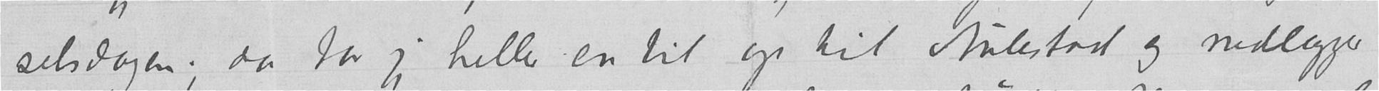selsdagen; da tar jeg heller en bil op til Aulestad og nedlegger
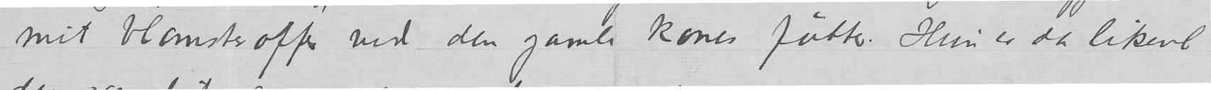mit blomsteroffer ved den gamle kones føtter. Hun er da likevel
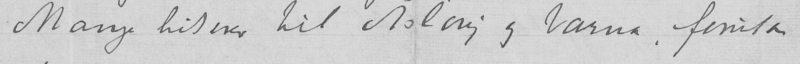Mange hilsener til Åslaug og barna, foruten
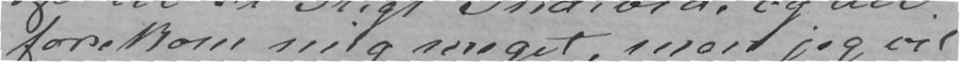forekom mig meget, men jeg vil
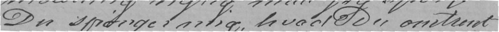Du spørger mig hvad Du omtrent
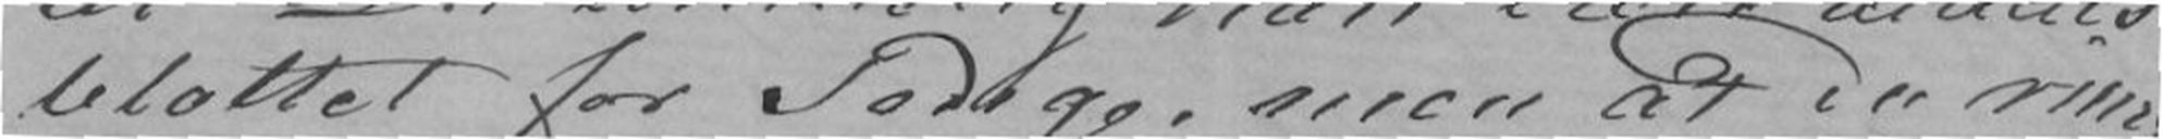blottet for Penge, men at Du rime-
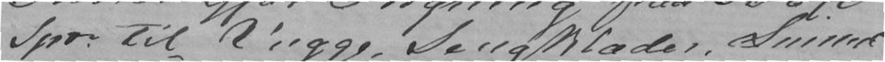Spdo til Vugge, Sengeklæder, Linned
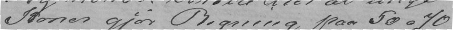Koner gjør Regning paa 50 a 70
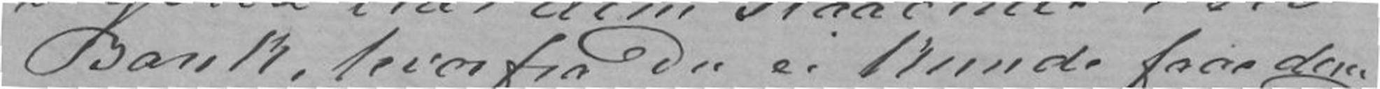Bank, hvorfra Du ei kunde faae dem
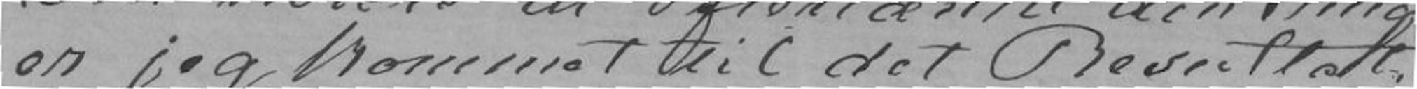er jeg kommet til det Resultat,
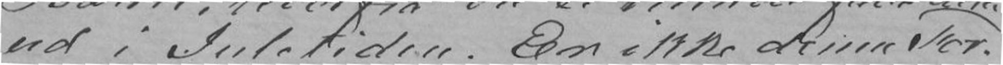ud i Juletiden. Er ikke denne For-
